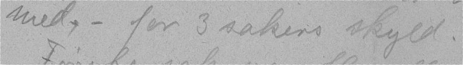med, - for 3 sakers skyld.
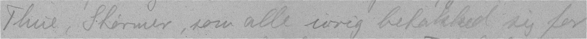Thue, Størmer, som alle ivrig betakked sig for
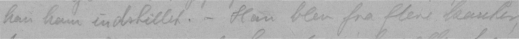han ham indstillet. - Han blev fra flere kanter,
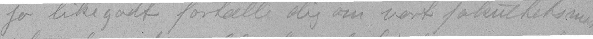jo likegodt fortælle dig om vort fakultetsmøte
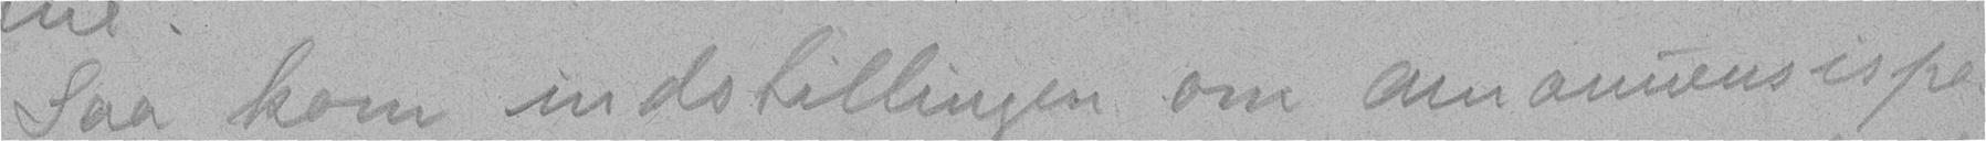Saa kom indstillingen om amanuensispo-
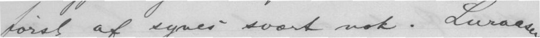først af synes svært nok. Luraasen
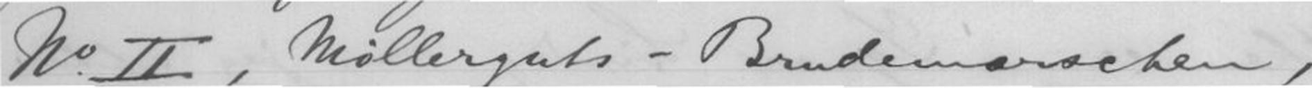N. II, Møllerguts-Brudemarchen,
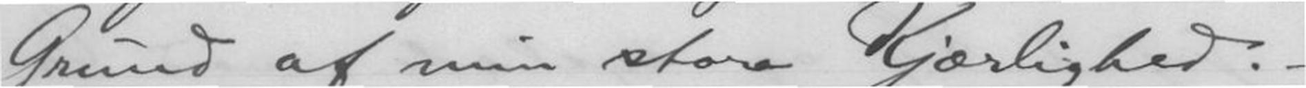Grund af min store Kjærlighed. -
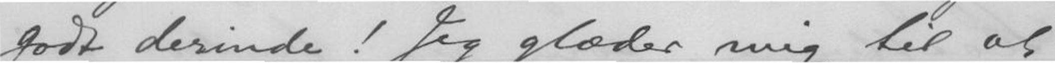godt derinde! Jeg glæder mig til at
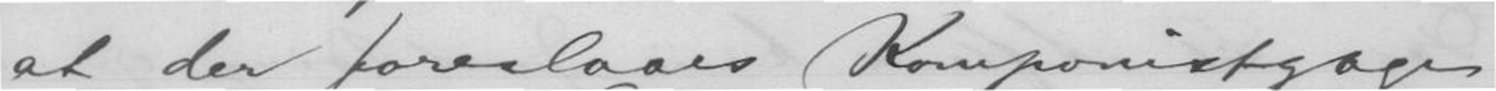at der foreslaaes Komponistgage
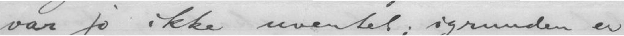var jo ikke uventet; igrunden er
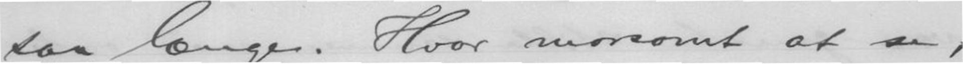saa længe. Hvor morsomt at se,
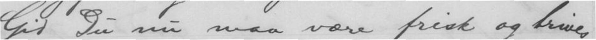Gid Du nu maa være frisk og trives
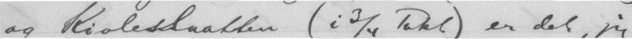og Kivleslaatten (i 3/4 Takt) er det, jeg
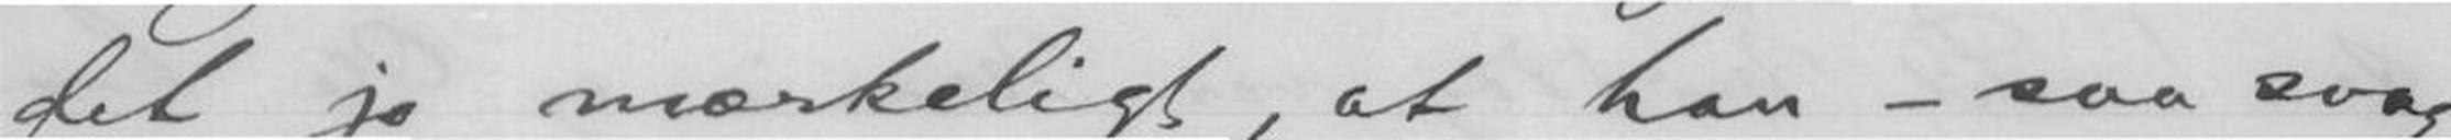det jo mærkeligt, at han - saa svag
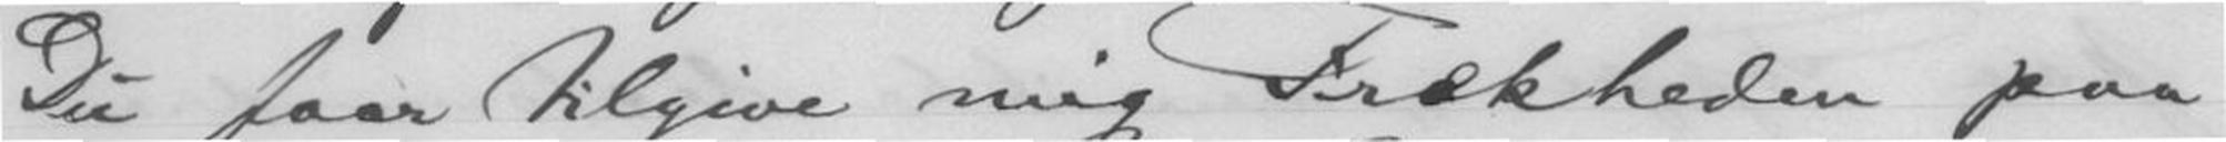Du faar tilgive Mig Frækheden paa
